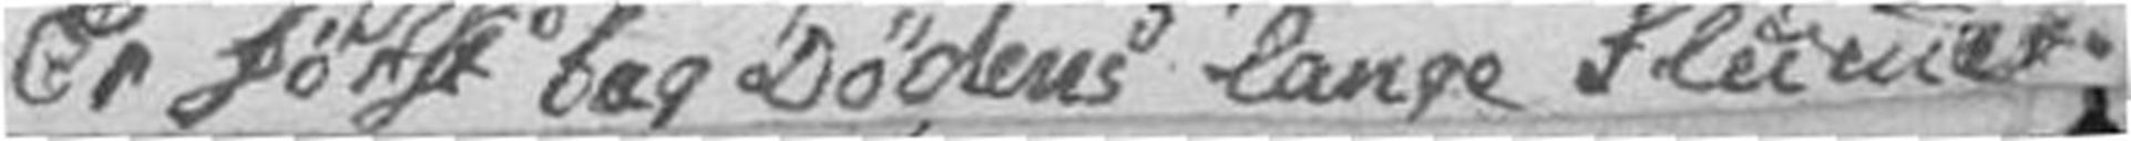Er først bag Dødens lange Slummer.
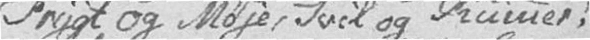Frygt og Møje, Tvil og Kummer!
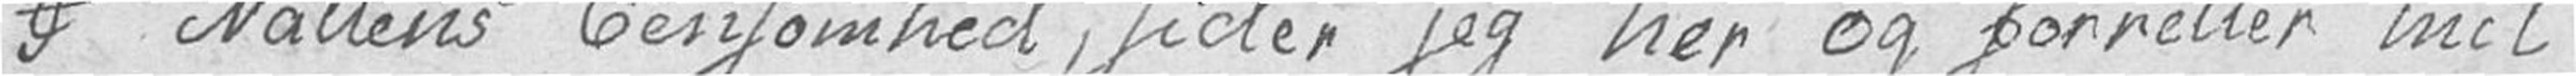I Nattens Eensomhed, sider jeg her og forretter mit
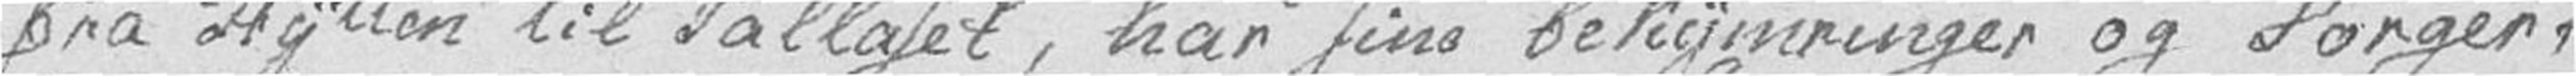fra Hytten til Pallaset, har sine bekymringer og Sorger,
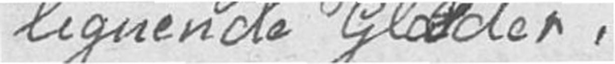lignende Glæder.
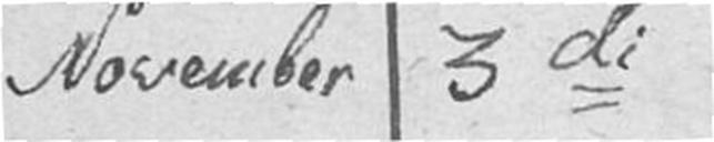November 3di
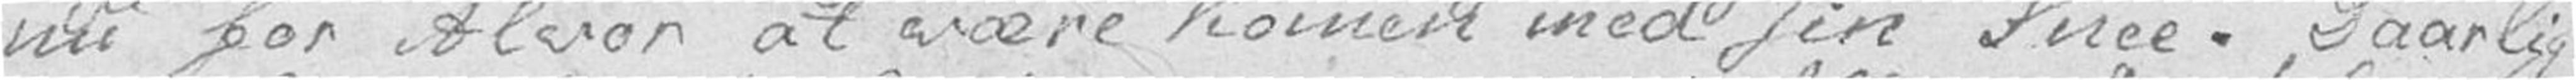nu for Alvor at være kommen med sin Snee. Daarlig
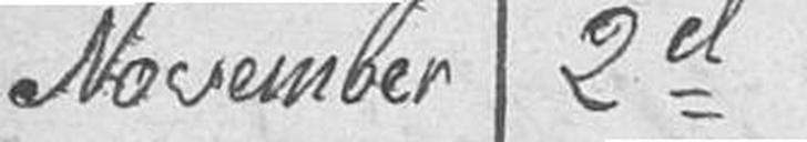November 2d
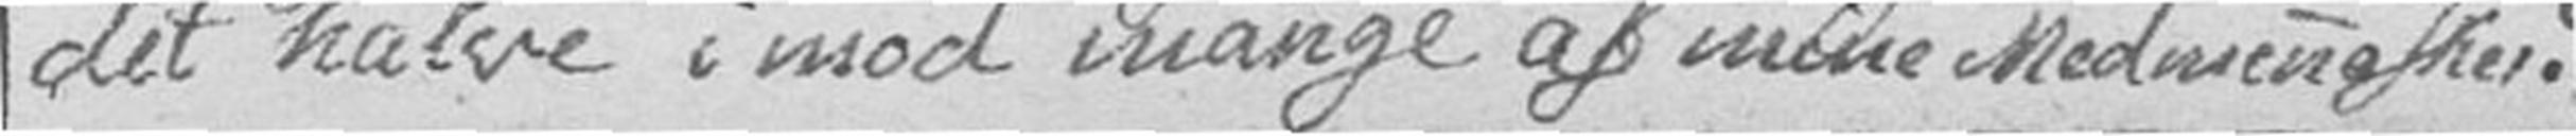det halve i mod mange af mine Medmennesker.
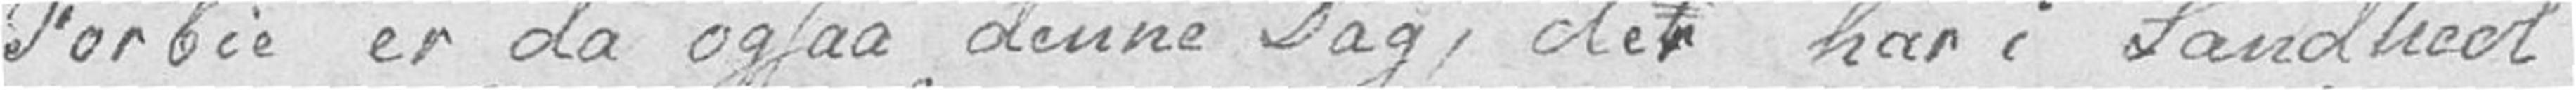Forbie er da ogsaa denne Dag, der har i Sandhed
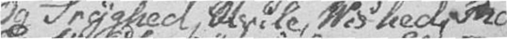Og Tryghed, Hvile, Vished, Ro
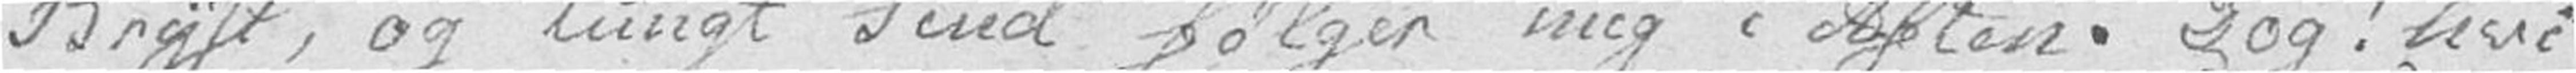Bryst, og tungt Sind følger mig i Aften. Dog! hvi
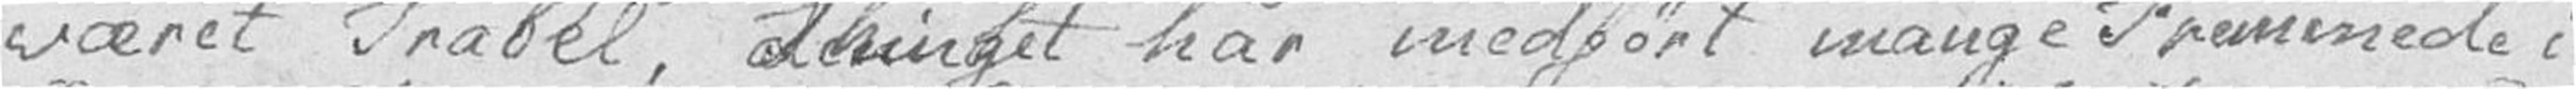været Trabel. Thinget har medført mange Fremmede i
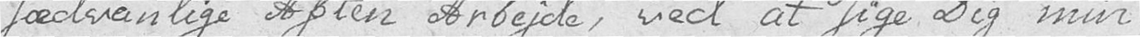sædvanlige Aften Arbejde, ved at sige Dig min
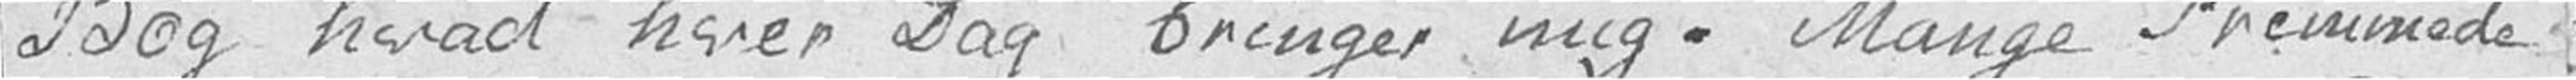Bog hvad hver Dag bringer mig. Mange Fremmede
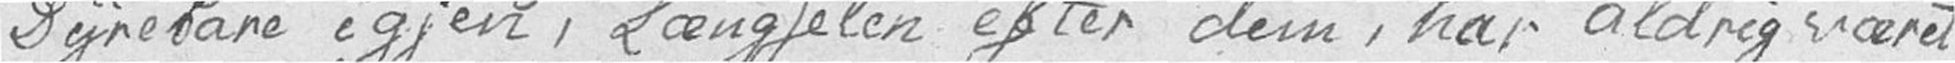Dyrebare igjen, Længselen efter dem, har aldrig været
